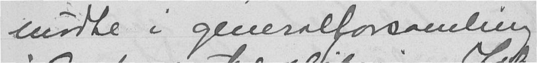mødte i generalforsamling
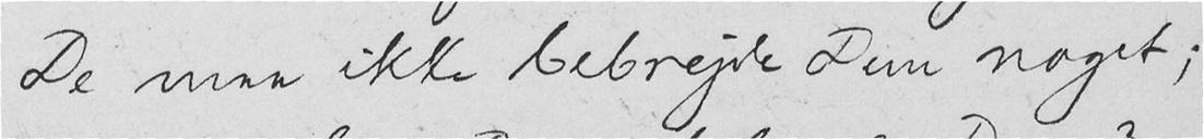De maa ikke bebrejde Dem noget;
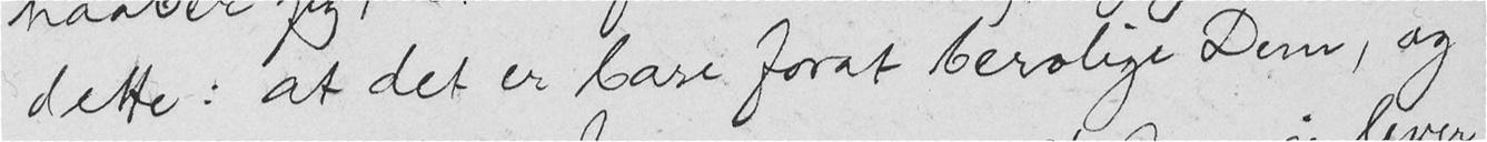dette: at det er bare forat berolige Dem, og
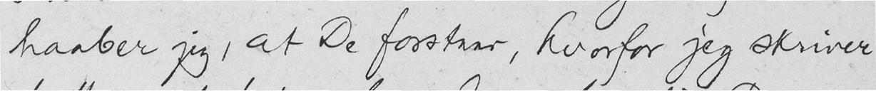haaber jeg, at De forstaar, hvorfor jeg skriver
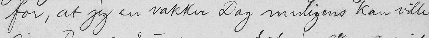for, at jeg en vakker Dag muligens kan ville
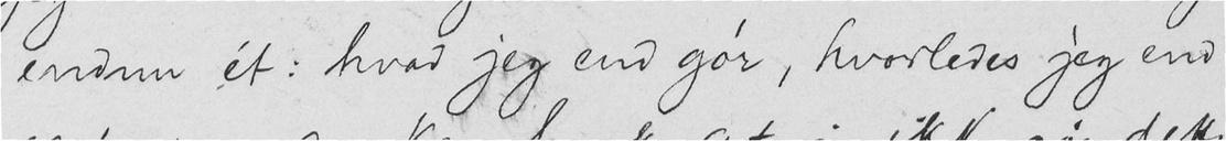endnu ét: hvad jeg end gør, hvorledes jeg end
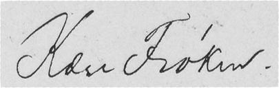Kære Frøken.
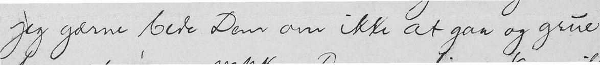jeg gærne bede Dem om ikke at gaa og grue
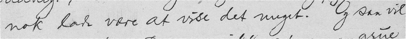nok lade være at vise det meget. Og saa vil
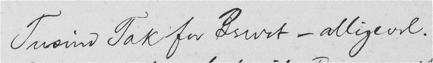Tusind Tak for Brevet - alligevel.
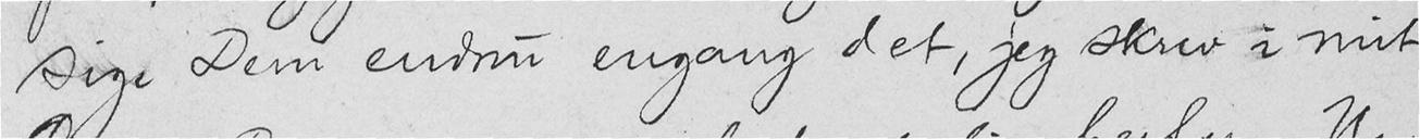sige Dem endnu engang det, jeg skrev i mit
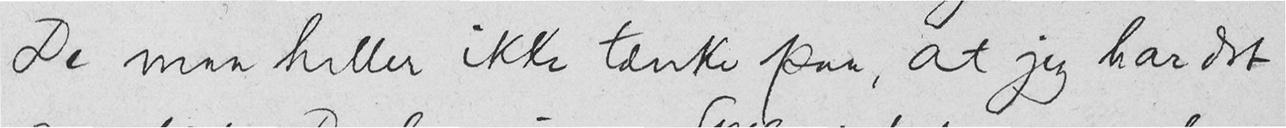De maa heller ikke tænke paa, at jeg har det
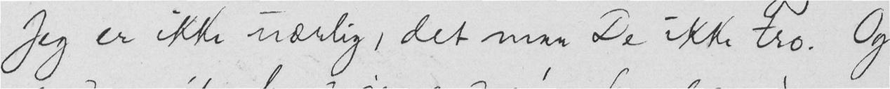Jeg er ikke uærlig, det maa De ikke tro. Og
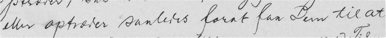eller optræder saaledes forat faa Dem til at
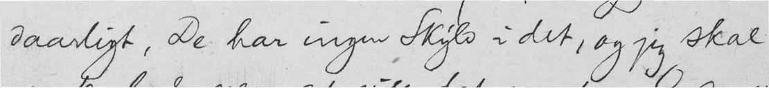daarligt, De har ingen Skyld i det, og jeg skal
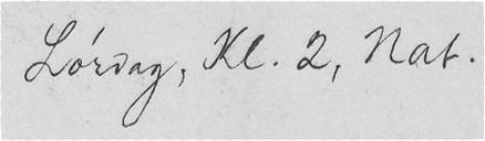Lørdag, Kl. 2, nat.
